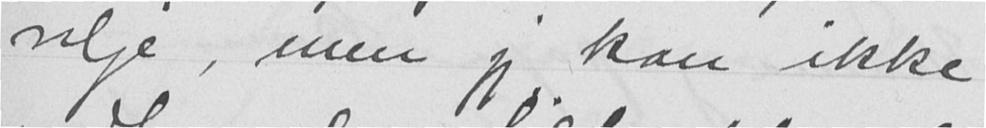vilje, men jeg kan ikke
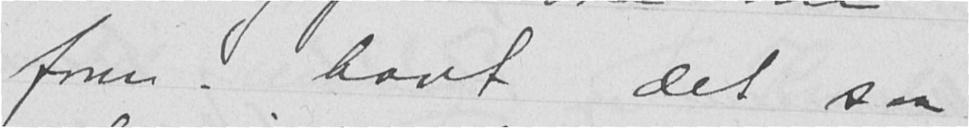form. havt det saa
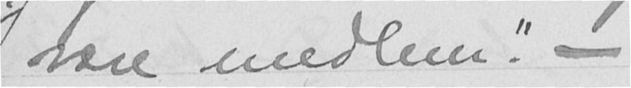være medlem." -
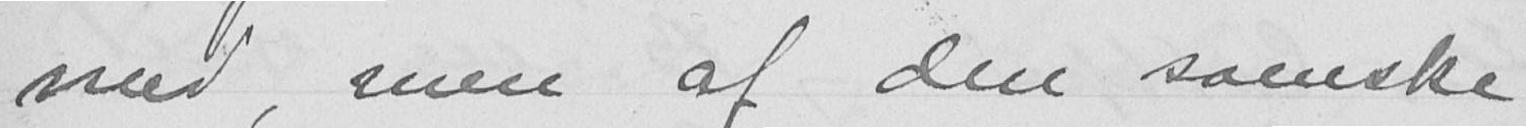med, men af den svenske
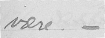være. -
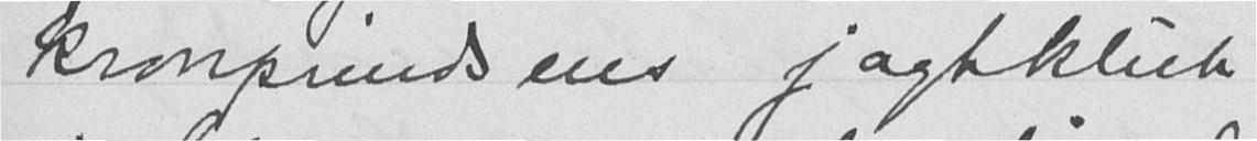kronprindsens jagtklub
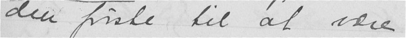den første til at være
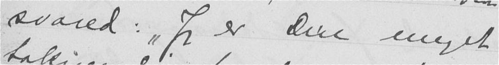svared: "Jeg er Dere meget
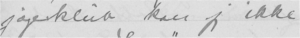jægerklub kan jeg ikke
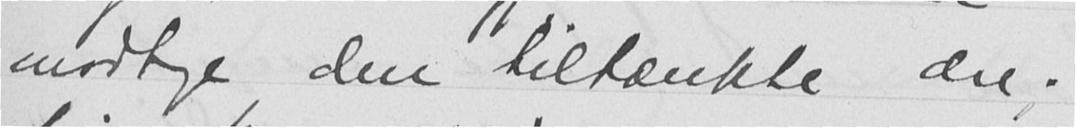modtage den tiltænkte ære;
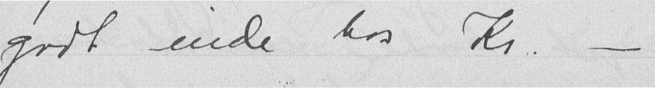godt inde hos Kr. -
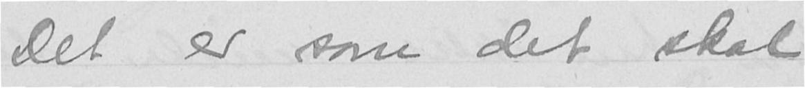Det er som det skal
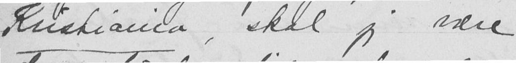Kristiania, skal jeg være
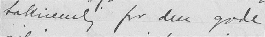taknemlig for den gode
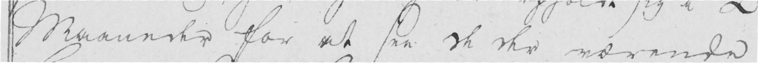Maaneder for at see de der værende
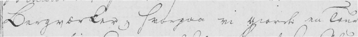Bergværker; hvorpaa vi giorde en Tur
